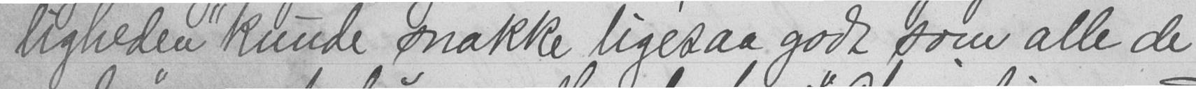ligheden "kunde snakke ligesaa godt som alle de
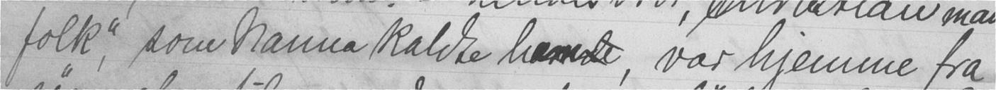folk", som Nanna kaldte hende, var hjemme fra
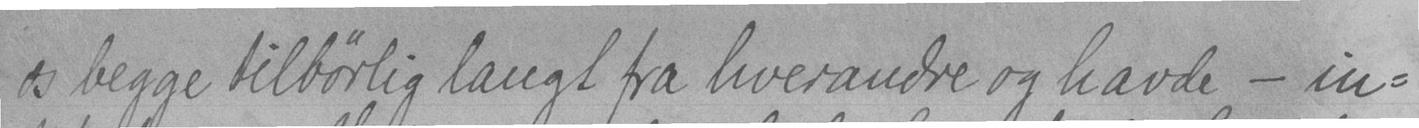os begge tilbørlig langt fra hverandre og havde - in-
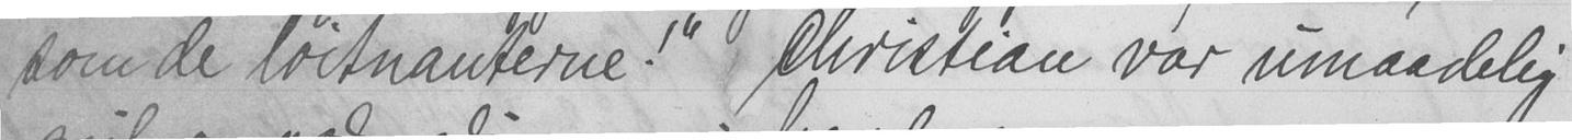som de løitnanterne!" Christian var umaadelig
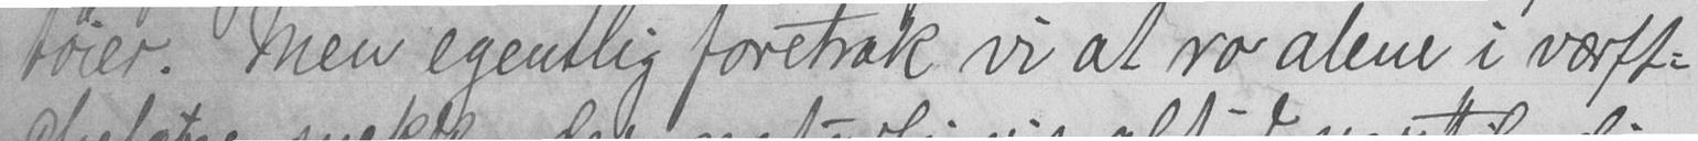tøier. Men egentlig foretrak vi at ro alene i værft-
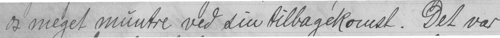os meget muntre ved sin tilbagekomst. Det var
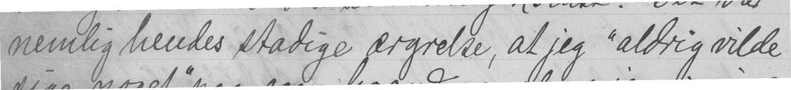nemlig hendes stadige ærgrelse, at jeg "aldrig vilde
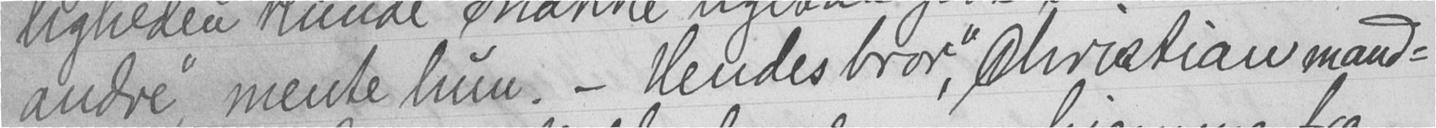andre," mente hun. - Hendes bror, "Christian mand-
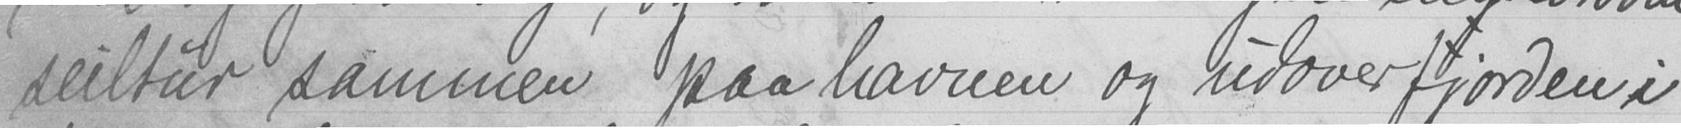seiltur sammen paa havnen og udover fjorden i
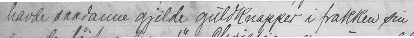havde saadanne gjilde guldknapper i frakken sin
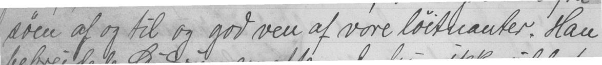søen af og til og god ven af vore løitnanter. Han
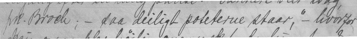frk. Broch; - saa deiligt poteterne staar," - hvorfor
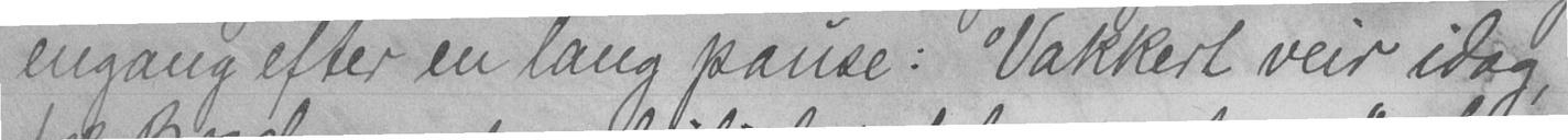engang efter en lang pause: "Vakkert veir idag,
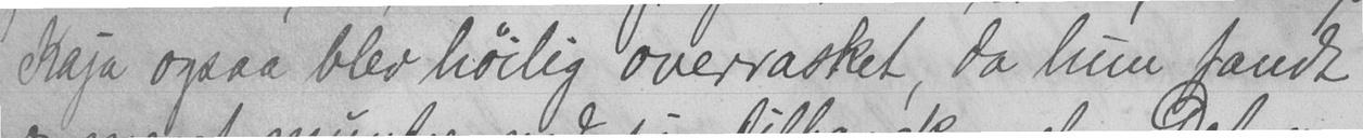Kaja ogsaa blev høilig overrasket, da hun fandt
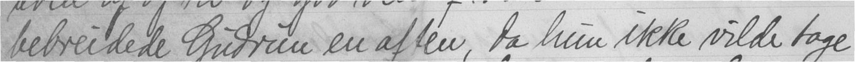bebreidede Gudrun en aften, da hun ikke vilde tage
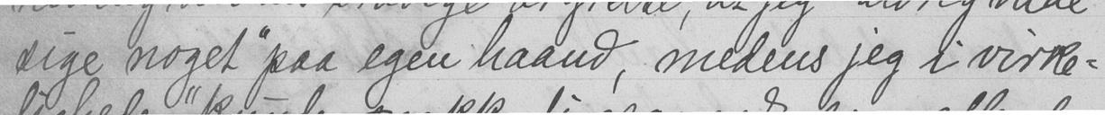sige noget "paa egen haand, medens jeg i virke-
